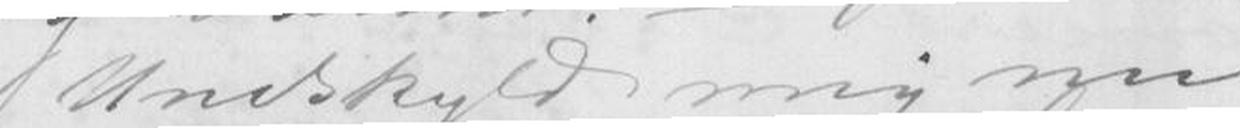Undskyld mig nu
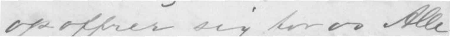opoffrer sig for os Alle.
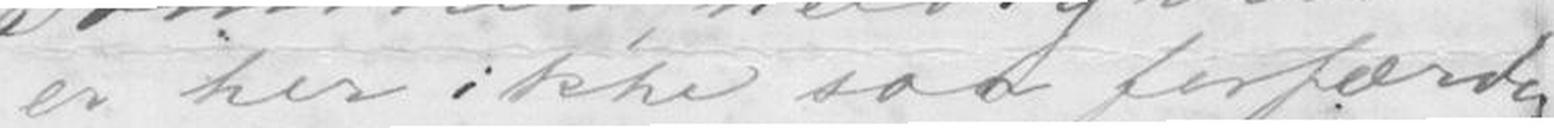er her ikke saa forfærde-
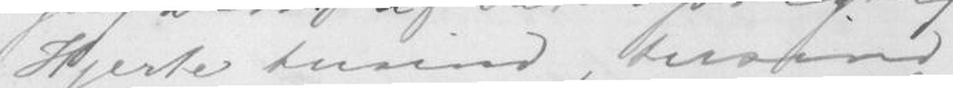Hjerte tusind, tusind
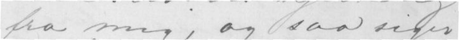fra mig, og saa siger
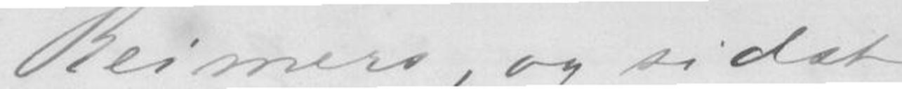Reimers, og sidst
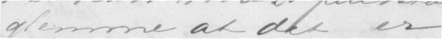glemme at det er
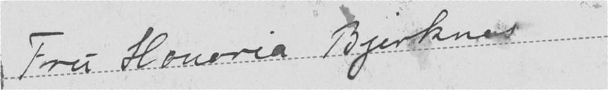Fru Honoria Bjerknes
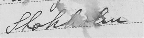Stokholm
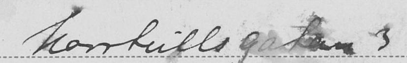Norrtullsgatan 3
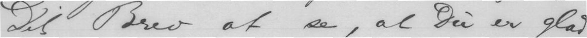Dit Brev at se, at Du er glad
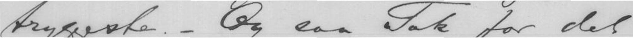tryggeste. - Og saa tak for det
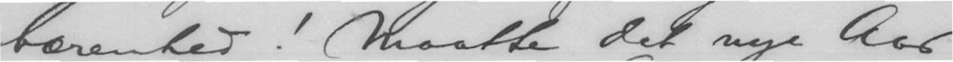bærenhed! Maatte det nye Aar
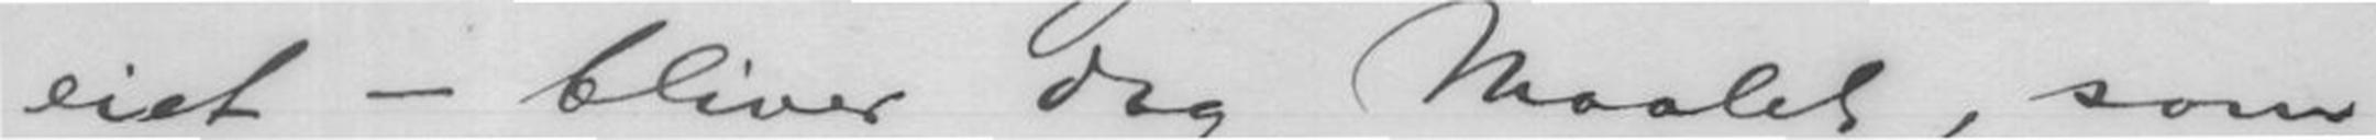eiet - bliver dog Maalet, som
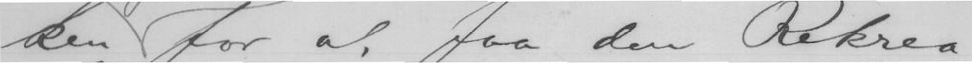ken for at faa den Rekrea-
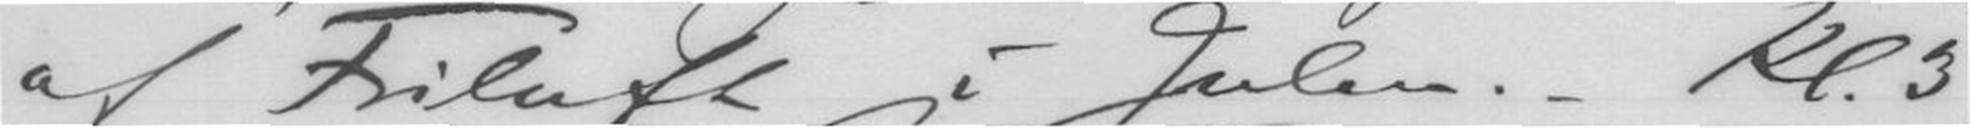af Friluft i Julen. - Kl.3
